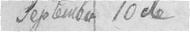September 10de
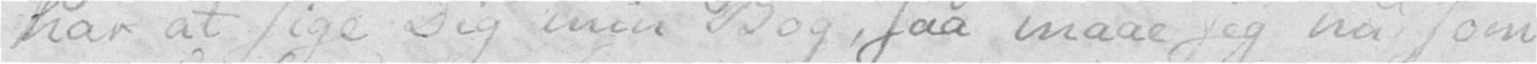har at sige Dig min Bog, saa maae jeg nu som
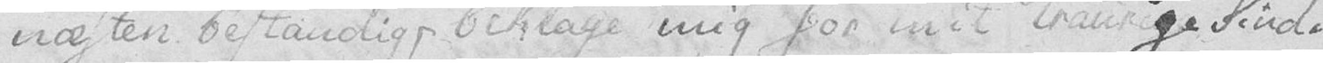næsten bestandig, beklage mig for mit traurige Sind.
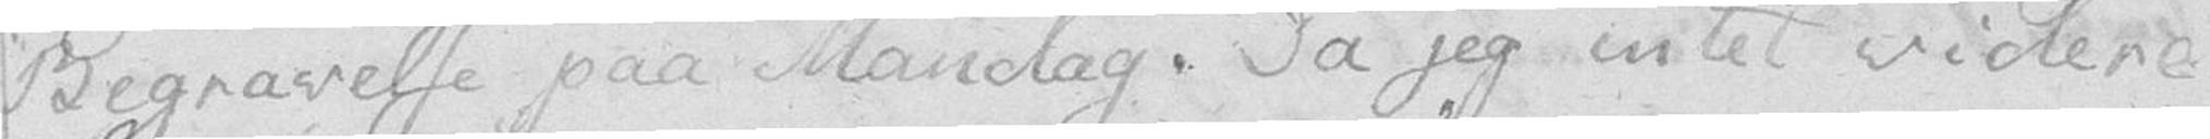Begravelse paa Mandag. Da jeg intet videre
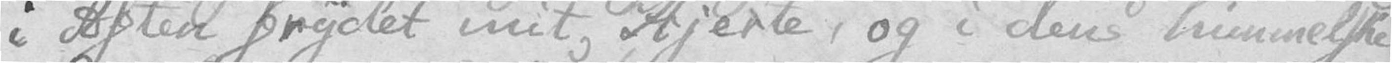i Aften frydet mit Hjerte, og i dens himmelske
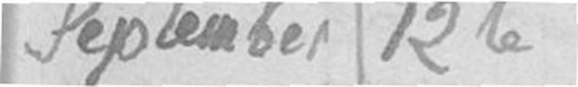September 12te
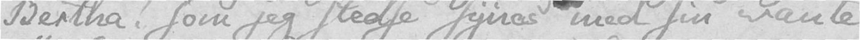Bertha! som jeg stedse synes med sin vante
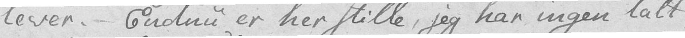lever. – Endnu er her stille, jeg har ingen talt
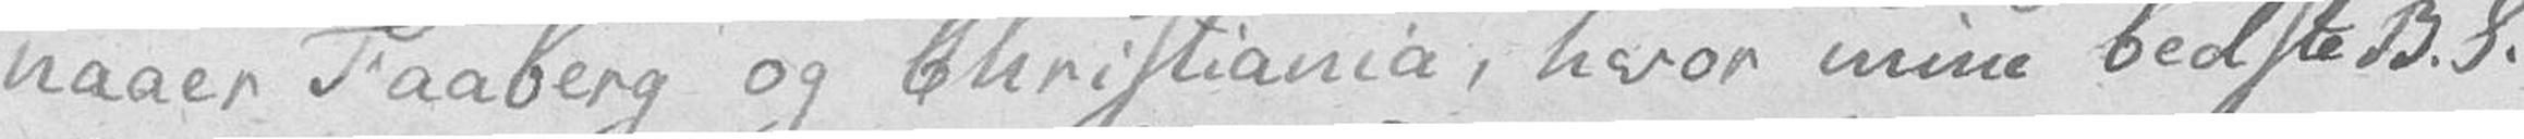naaer Faaberg og Christiania, hvor mine bedste B. S.
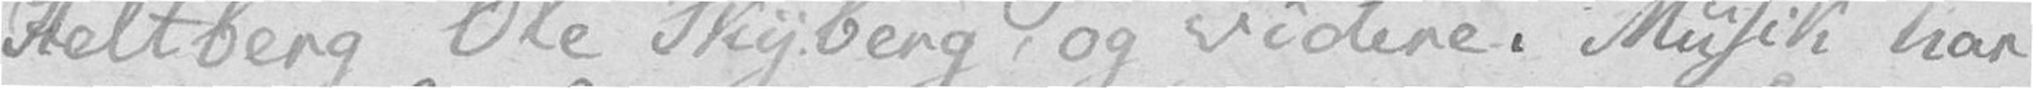Heltberg Ole Skyberg, og videre. Musik har
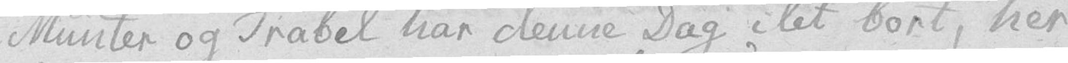Munter og Trabel har denne Dag ilet bort, her
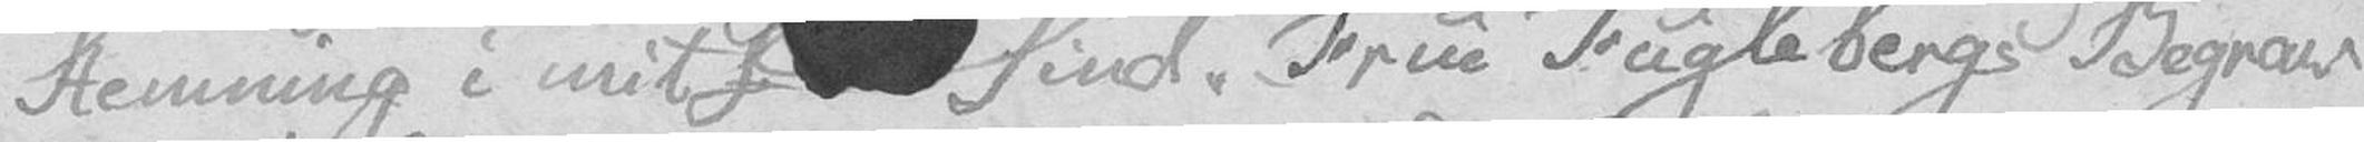Stemning i mit  Sind. Frue Fuglebergs Begrav
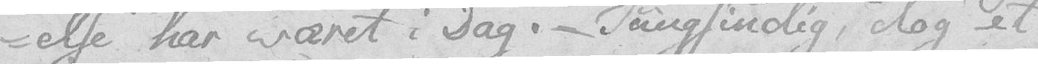-else har været i Dag. – Tungsindig, dog et
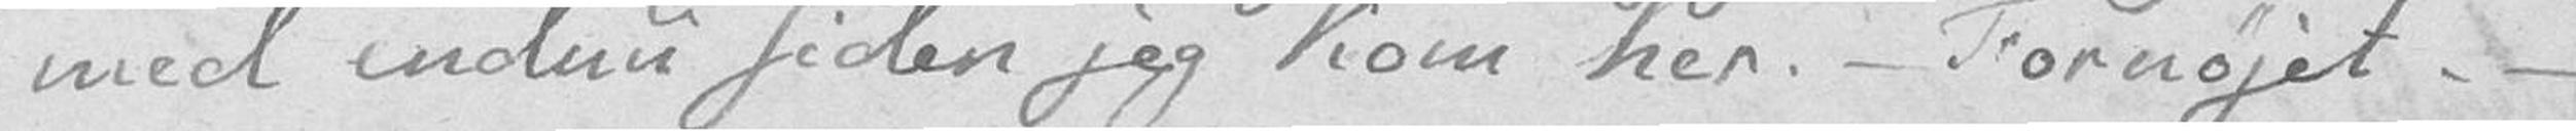med endnu siden jeg kom her. – Fornøjet. –
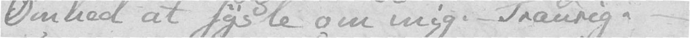Ømhed at sysle om mig. – Traurig. –
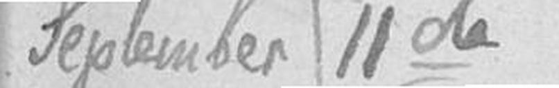September 11de
